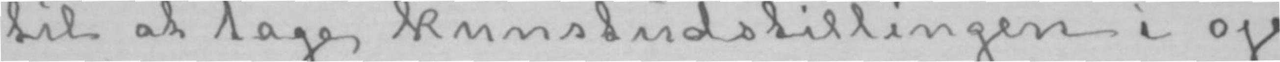til at tage kunstudstillingen i øje-
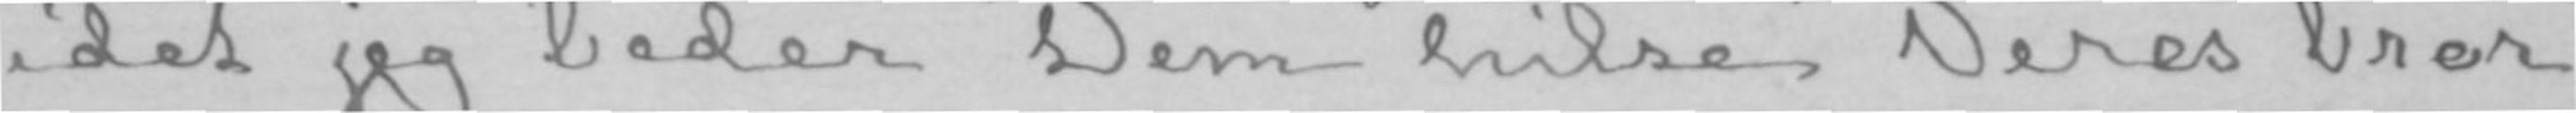idet jeg beder Dem hilse Deres bror
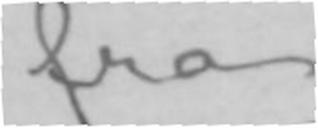fra
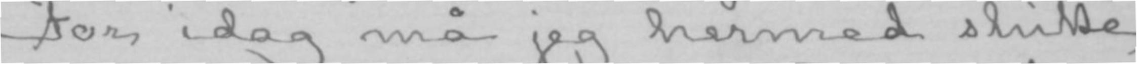For idag må jeg hermed slutte
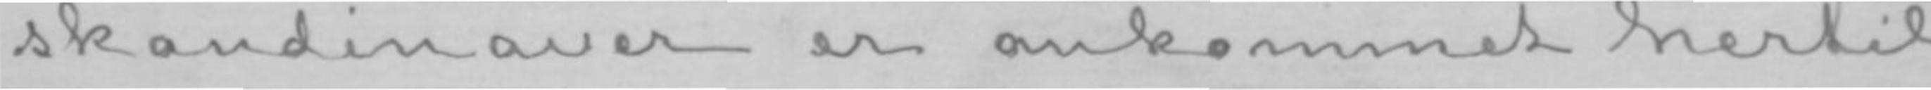skandinaver er ankommet hertil,
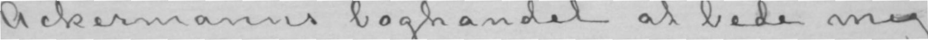Ackermanns boghandel at bede mig
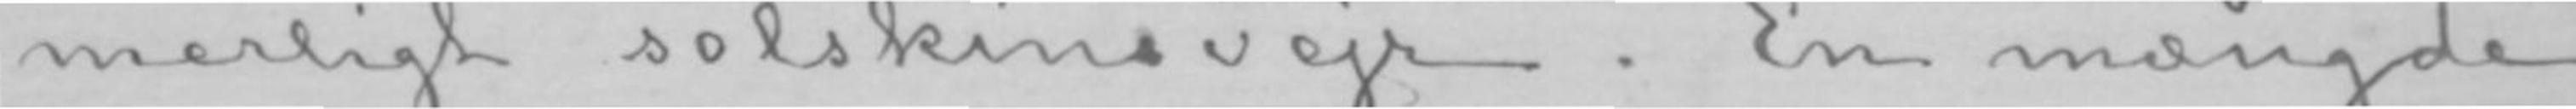merligt solskinsvejr. En mængde
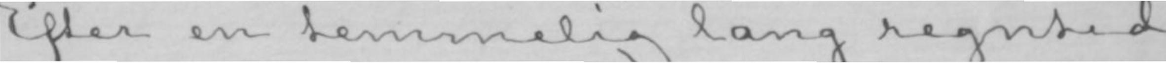Efter en temmelig lang regntid
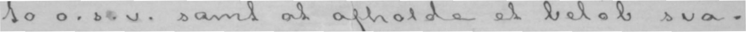to o. s. v. samt at afholde et beløb sva-
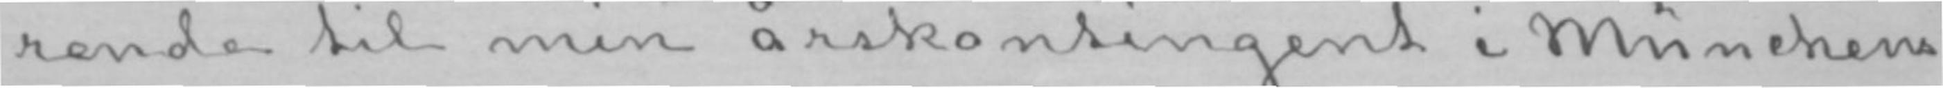rende til min årskontingent i Münchens
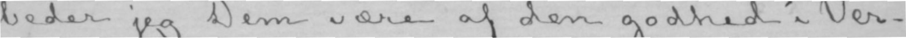beder jeg Dem være af den godhed i Ver-
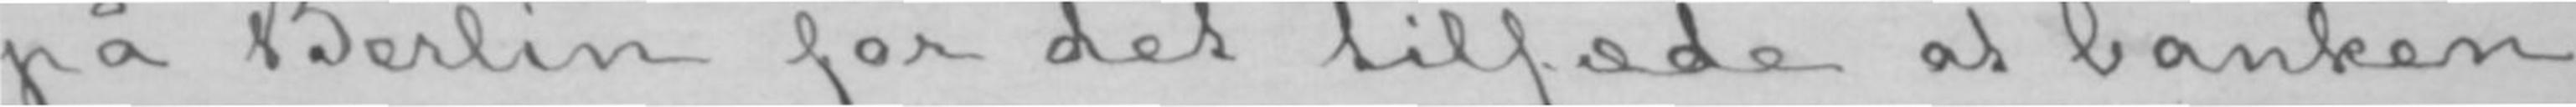på Berlin for det tilfæde at banken
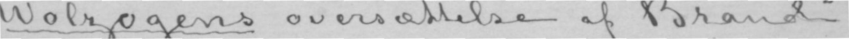Wolzogens oversættelse af «Brand»,
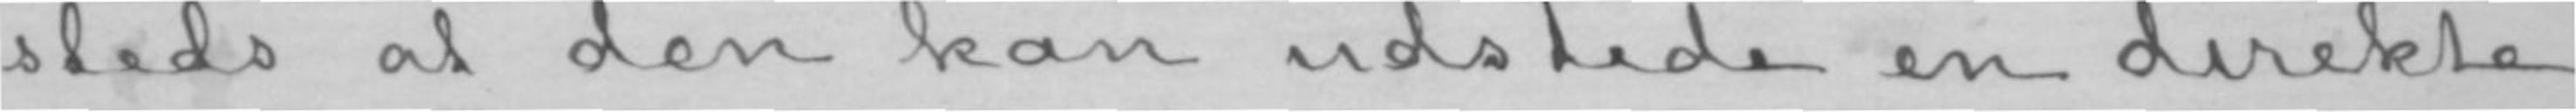steds at den kan udstede en direkte
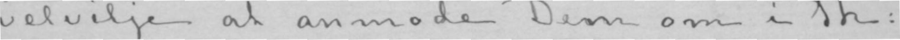velvilje at anmode Dem om i Th:
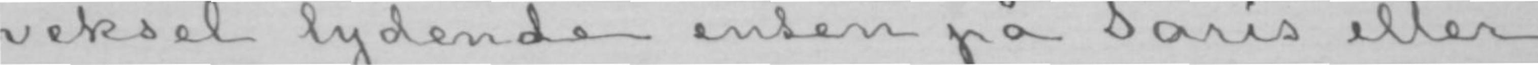veksel lydende enten på Paris eller
